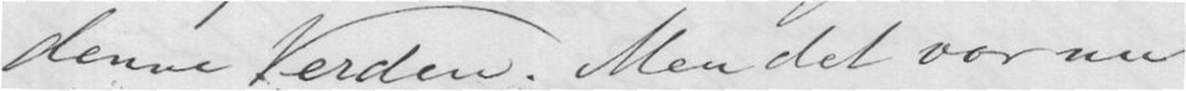denne Verden. Men det var nu
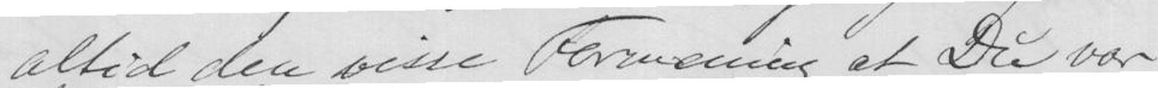altid den visse Formening at Du var
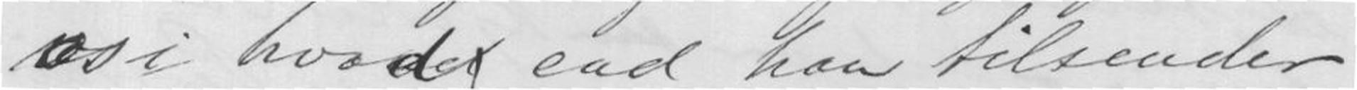os i hvad end han tilsender
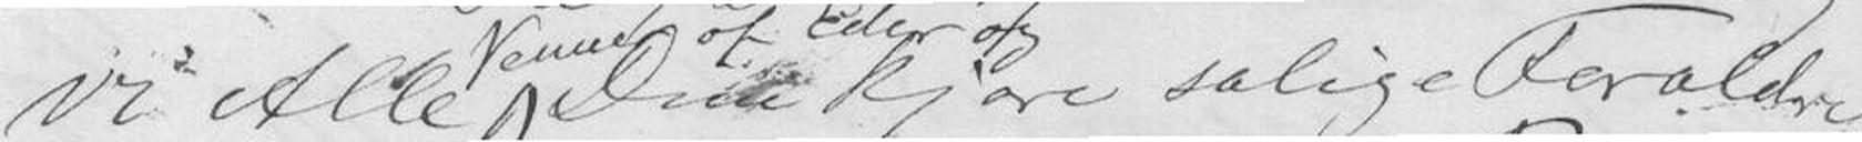vi Alle Dine kjære salige Forældre,
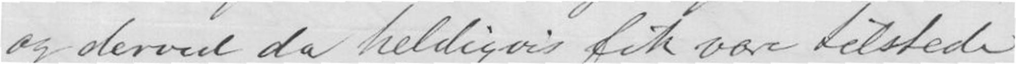og derved da heldigvis fik være tilstede
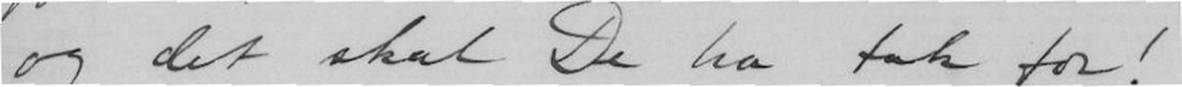og det skal De ha tak for! -
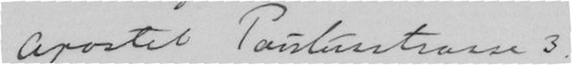Apostel Paulusstrasse 3.
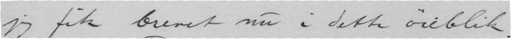jeg fik brevet nu i dette øieblik.
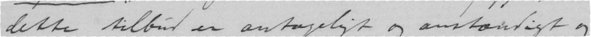dette tilbud er antageligt og anstændigt og
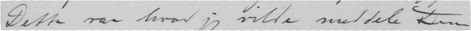Dette var hvad jeg vilde meddele Dem,
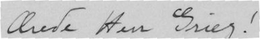Ærede Herr Grieg!
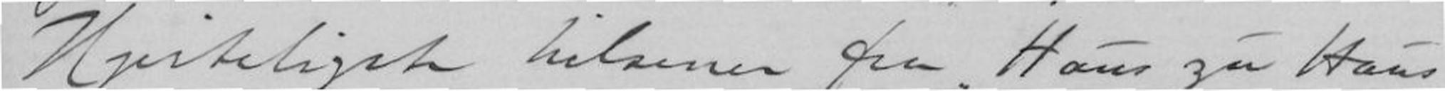Hjerteligste hilsener fra "Haus zu Haus
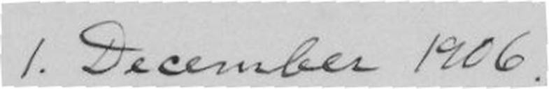1. December 1906.
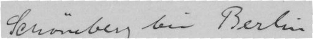Schöneberg bei Berlin
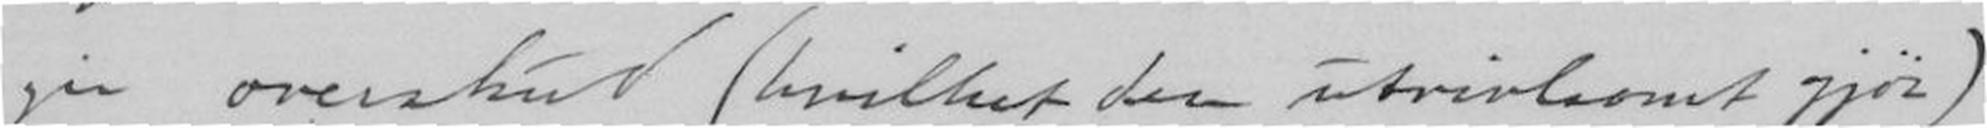gir overskud (hvilket den utvivlsomt gjør)
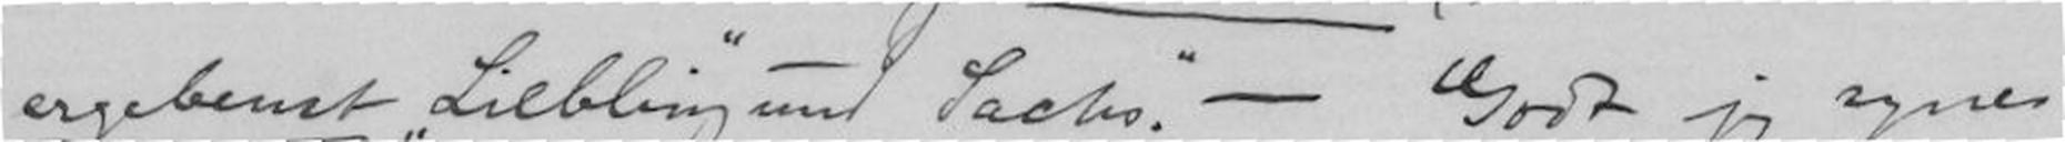ergebenst "Liebling und Sachs". - Godt, jeg synes
